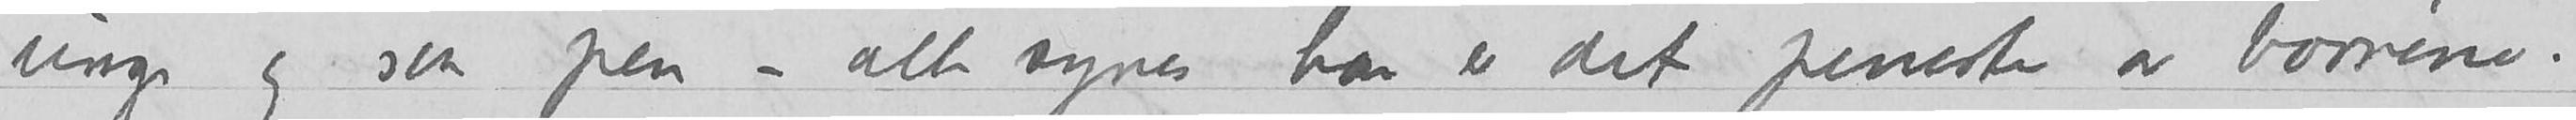unge og saa pen – alle synes han er det peneste av børnene.
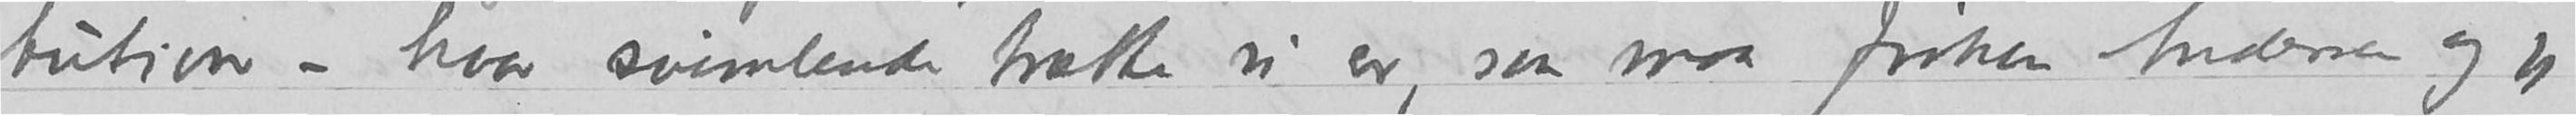tution – hvor svimlende trætte vi er, saa maa frøken Andersen og jeg
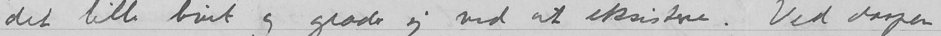det lille bunt og glæder sig ved at eksistere. Ved daapen
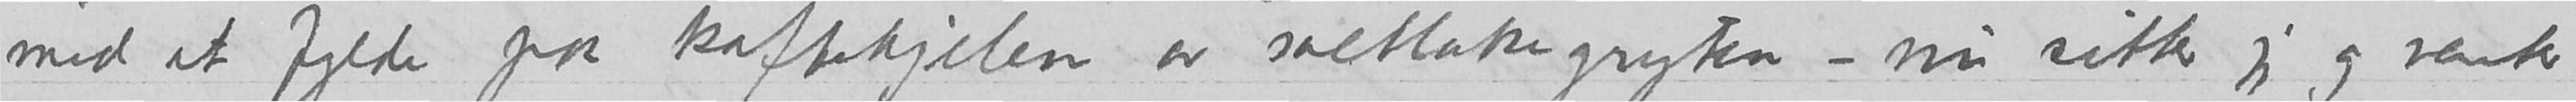med at fylde paa kaffekjelen av saltlakegryten – nu sitter jeg og venter
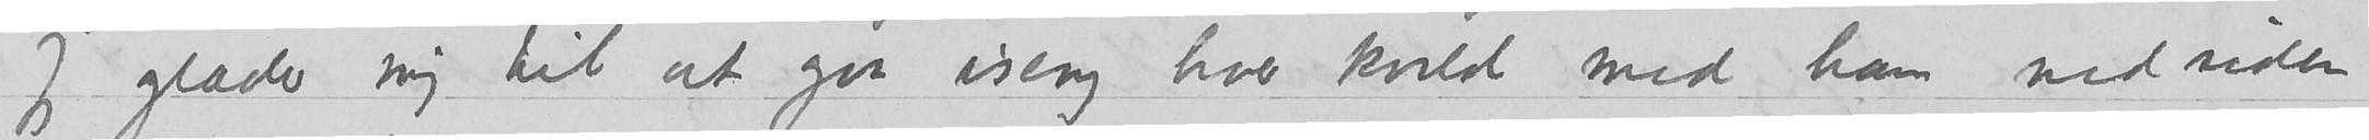Jeg glæder mig til at gaa iseng hver kveld med ham ved siden
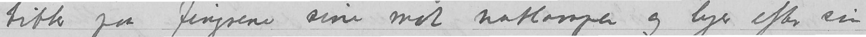titter paa fingrene sine mot natlampen og lyer efter sin
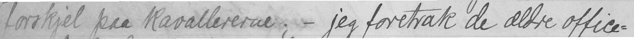forskjel paa kavallererne; - jeg foretrak de ældre office-
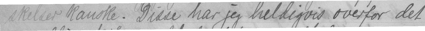skelser kanske. Disse har jeg heldigvis overfor det
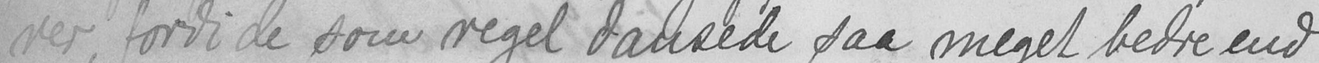rer, fordi de som regel dansede saa meget bedre end
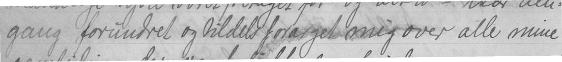gang forundret og tildels forarget mig over alle mine
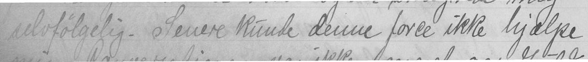selvfølgelig. Senere kunde denne force ikke hjælpe
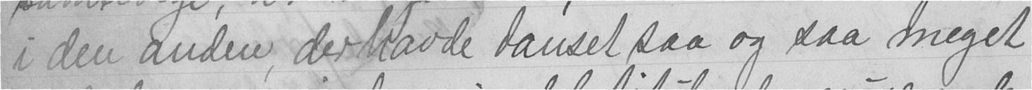i den anden, der havde danset saa og saa meget
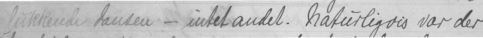lukkende dansen - intet andet. Naturligvis var der
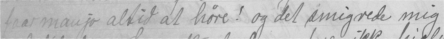faar man jo altid at høre! og det smigrede mig
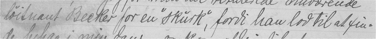løitnant Becker for en "skurk", fordi han lod til at fin-
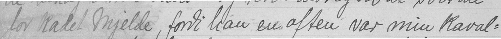for kadet Mjelde, fordi han en aften var min kaval-
